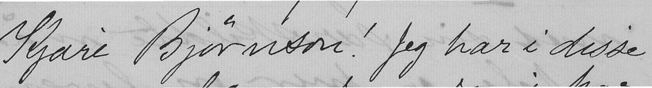Kjære Bjørnson! Jeg har i disse
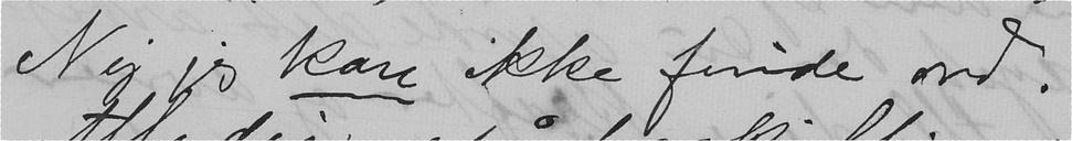Nej jeg kan ikke finde ord.
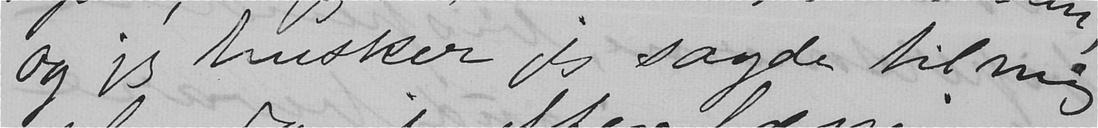og jeg husker jeg sagde til mig
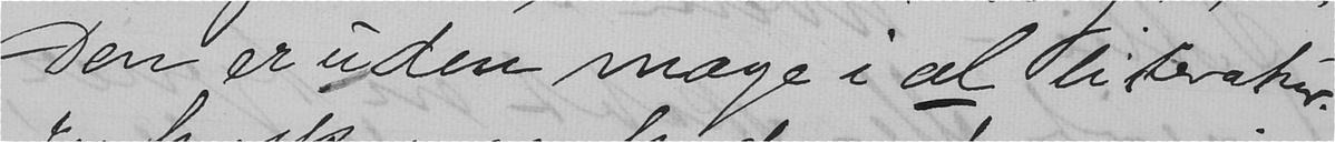Den er uden mage i al literatur.
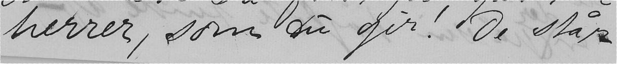herrer, som Du gir! De står
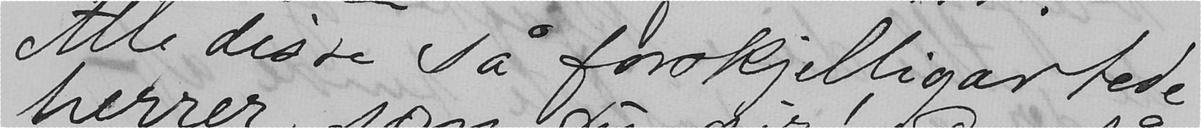Alle disse så forskjelligartede
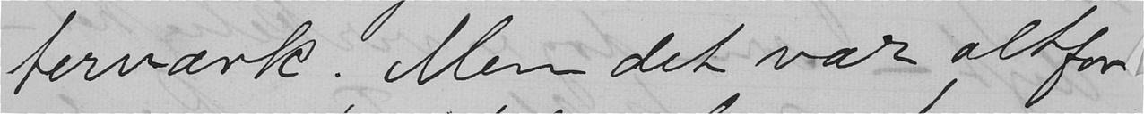terværk. Men det var altfor
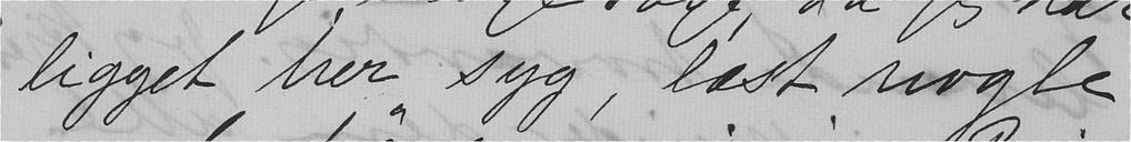ligget her syg, læst nogle
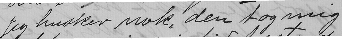Jeg husker nok, den tog mig
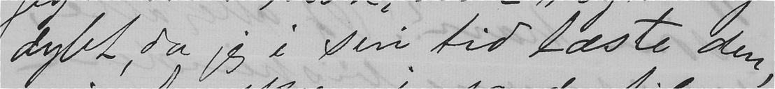dybt, da jeg i sin tid læste den,
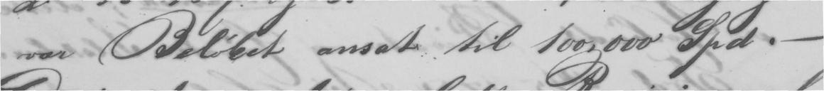var Beløbet ansat til 100,000 Spd. -
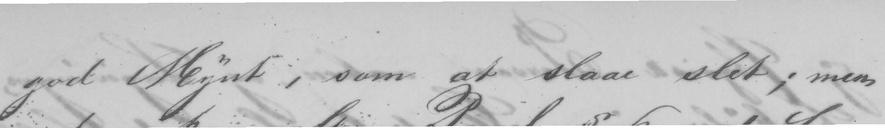god Mynt, som at slaae slet; men
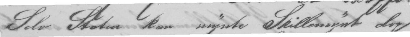Selv Staten kan mynte Skillemynt dog
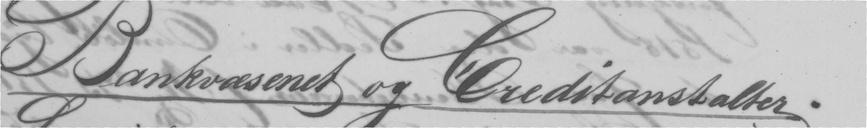Bankvæsenet og Creditanstalter.
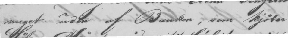meget uden af Banken, som kjøber
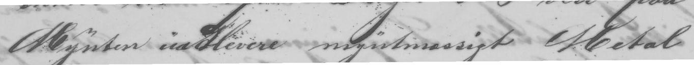Mynten indlevere myntmæssigt Metal
